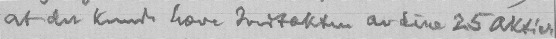at du kunde hæve Indtækten av dine 25 Aktier
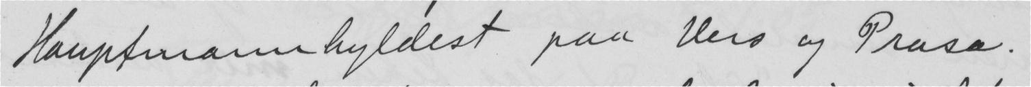Hauptmannhyldest paa Vers og Prosa.
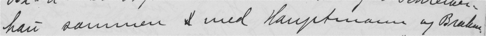hau sammen  med Hanptmann og Braben
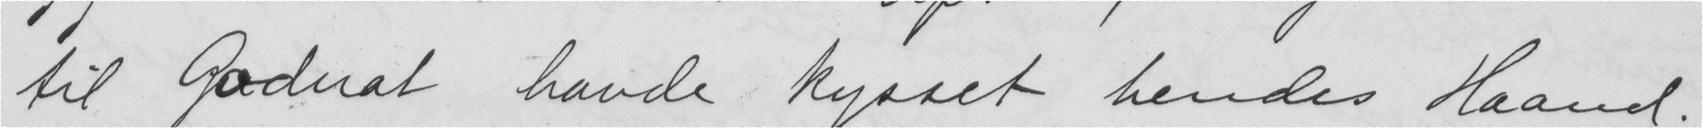til Godnat havde Rysset hendes Haand.
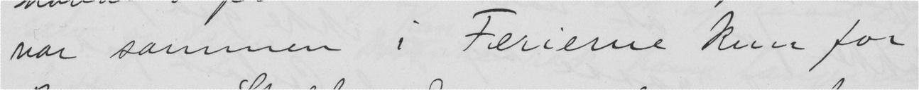var sammen i Ferierne kun for
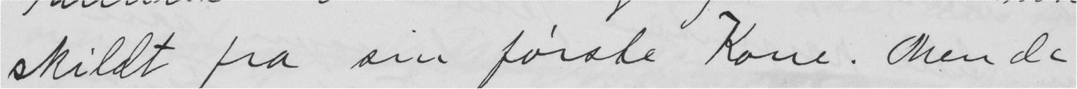skildt fra sin første Kone. Men de
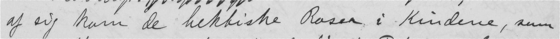af sig kom de hektiske Roser i Kindene, som
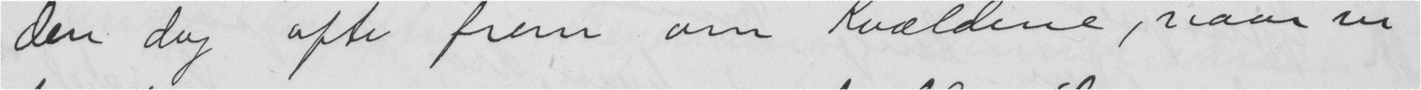den dag ofte frem om Kvældene, naar vi
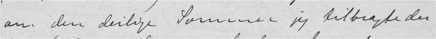om den deilige Sommer jeg tilbragte der
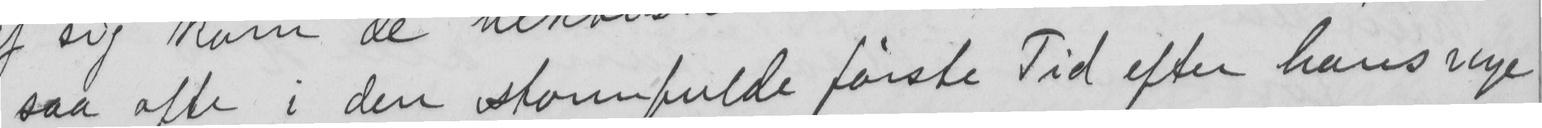saa ofte i den stormfulde første Tid efter hans nye
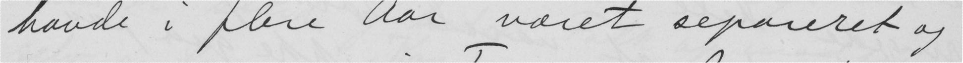havde i flere Aar været separeret og
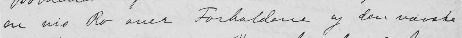en vis Ro over Forholdene og den værste
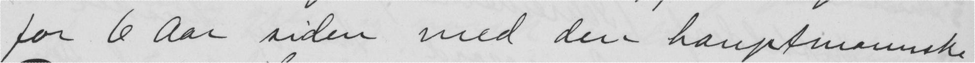for 6 Aar siden med den hauptmannske
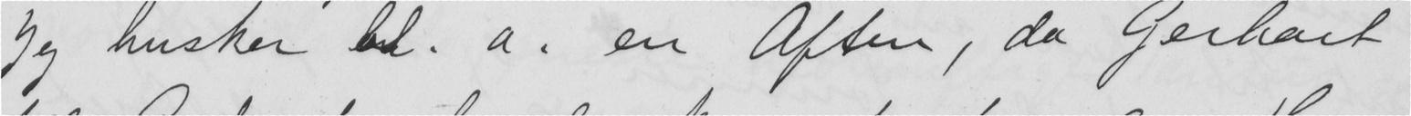Jeg husker bl. a. en Aften, da Gerhart
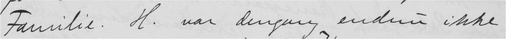Familie. H. var dengang endnu ikke
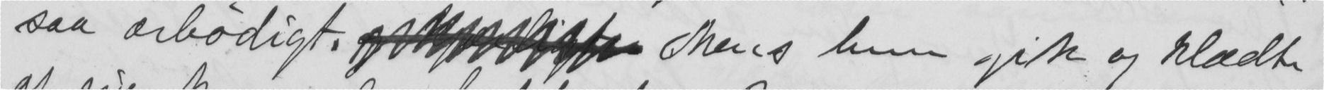saa ærbødigt.  Mens hun gik og klædte
